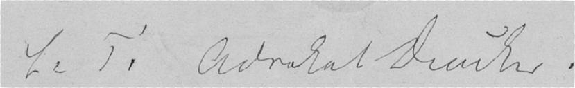S: T: Advocat Dunker
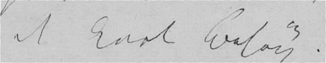et kort Besøg.
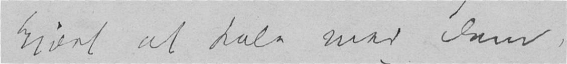kjært at tale med Dem,
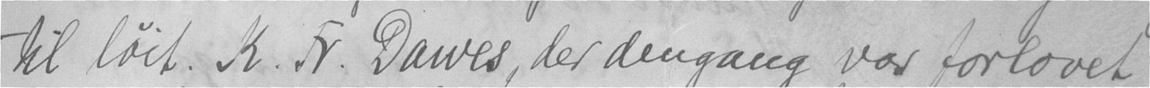til løit. K. Fr. Dawes, der dengang var forlovet
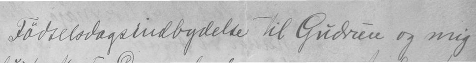Fødselsdagsindbydelse til Gudrun og mig
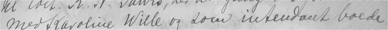med Karoline Wille og som intendant boede
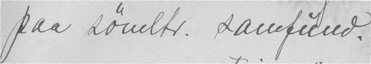paa sømlfr. samfund.
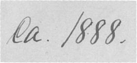Ca. 1888.
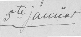5te januar
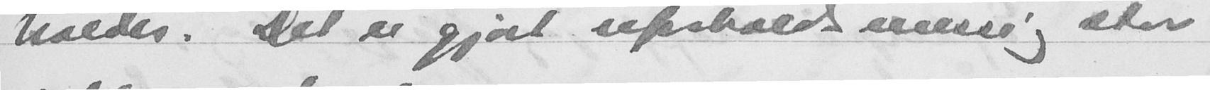holder. Det er gjort uforholdsmessig stor
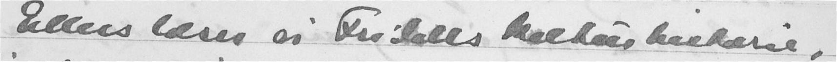Ellers læser vi Fridells koltug historie,
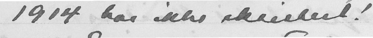1914 har ikke eksistert!
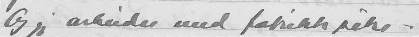Og jeg arbeider med fabrikkpike-
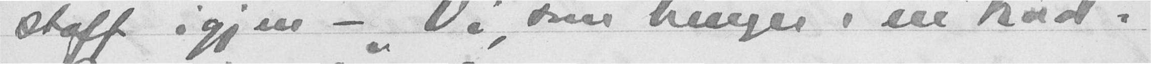stoff igjen - "Vi, som henger i en tråd."
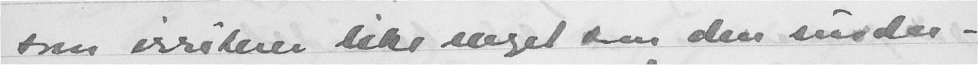som irriterer like meget som den under-
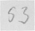53
